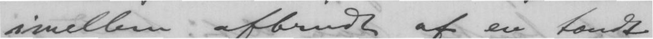imellem afbrudt af en tændt
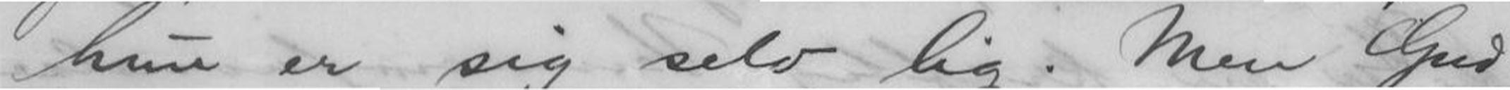hun er sig selv lig. Men Gud-
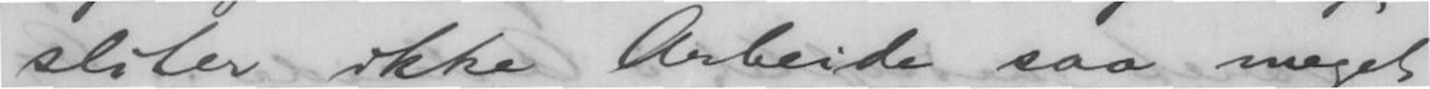sliter ikke Arbeide saa meget
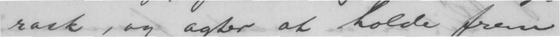rask, og agter at holde frem,
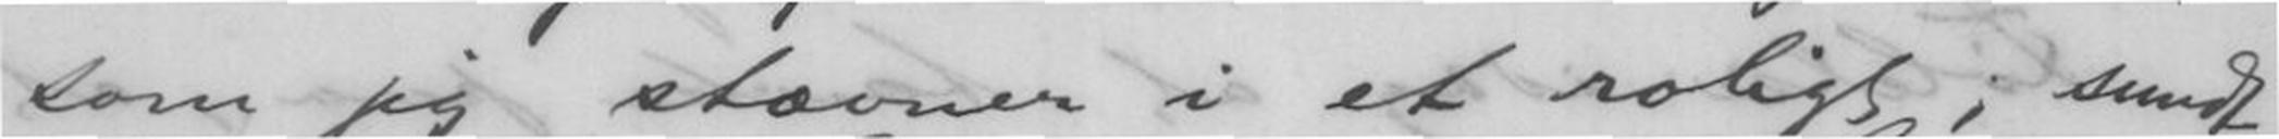som jeg stævner i et roligt, sundt
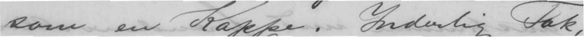som en Kappe. Inderlig Tak,
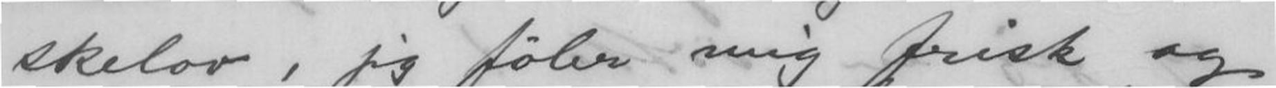skelov, jeg føler mig frisk og
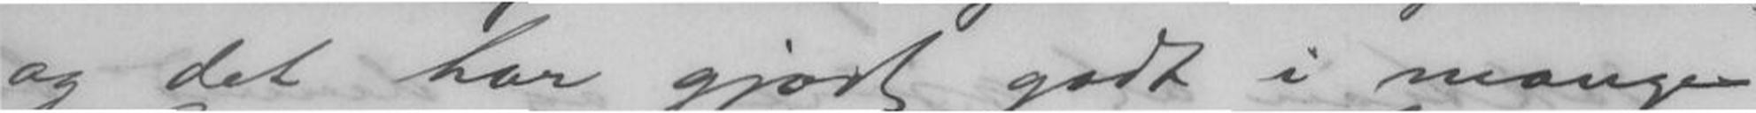og det har gjort godt i mange
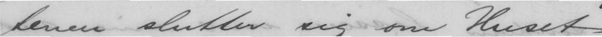tenen slutter sig om Huset
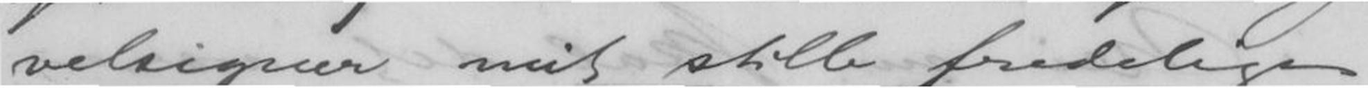velsigner mit stille fredelige
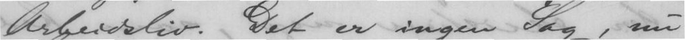Arbeidsliv. Det er ingen Sag, nu
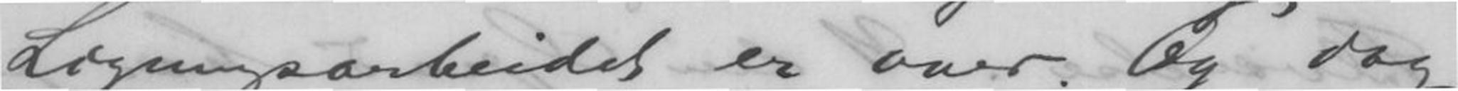Ligningsarbeidet er over. Og dog
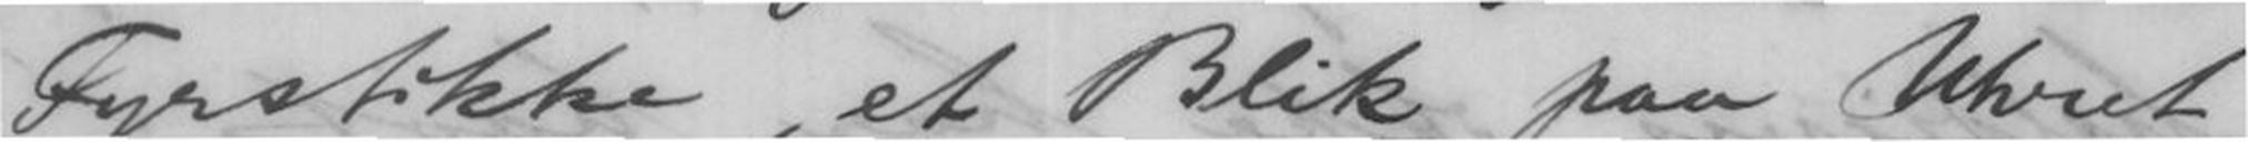Fyrstikke, et Blik paa Uhret
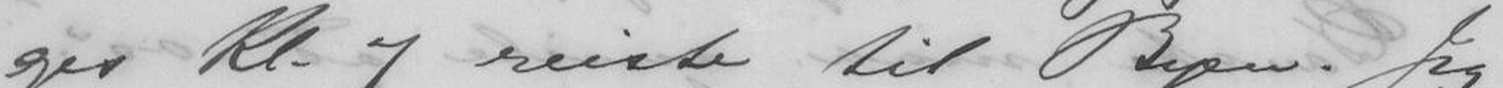ges Kl. 7 reiste til Byen. Jeg
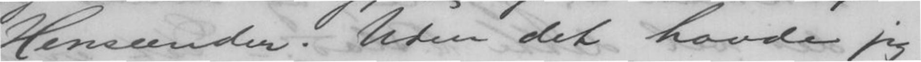Henseender. Uden det havde jeg
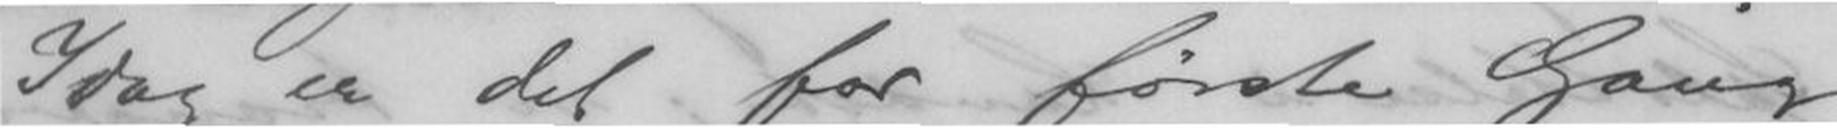Idag er det for første Gang
